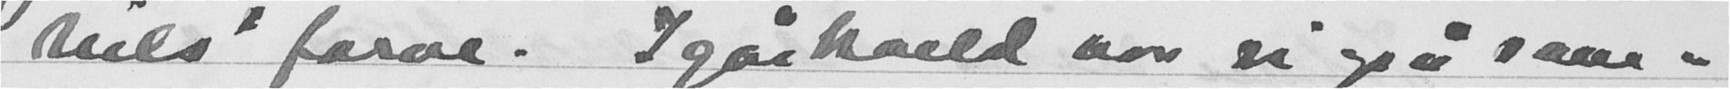Nils' farve. Igårkveld var vi også sam-
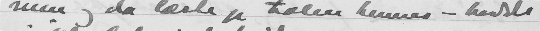men og da læste jeg talen hennes - hadde
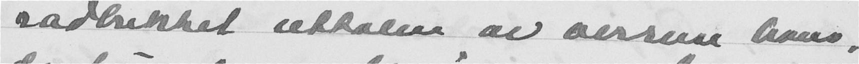radbrekket uttalen ev versene hans,
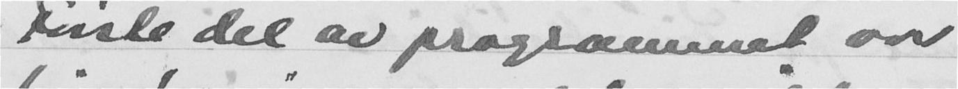Første del av programmet var
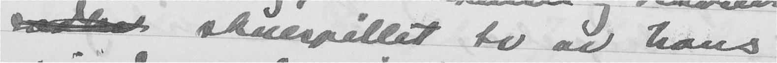skuespillet to av hans
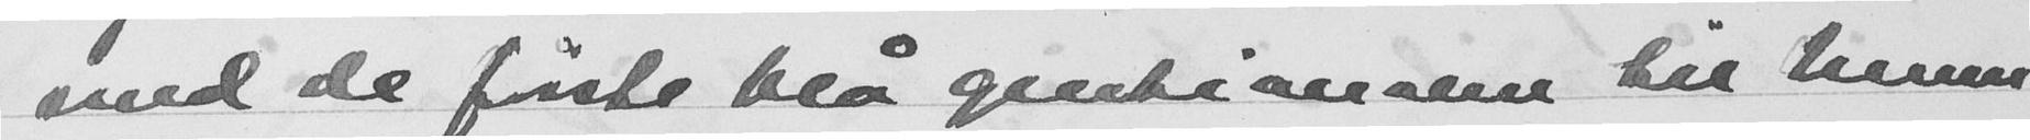med de friske blå gentianaene til henne
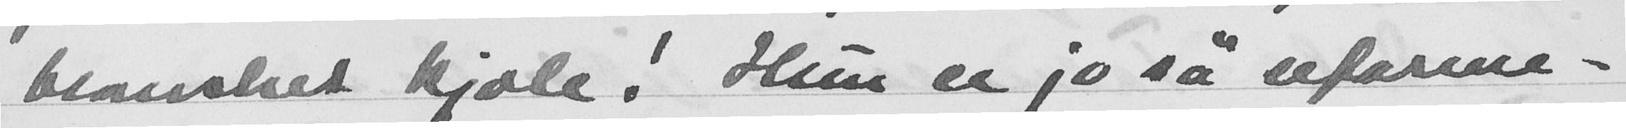blomseret kjole! Hun er jo så uforene-
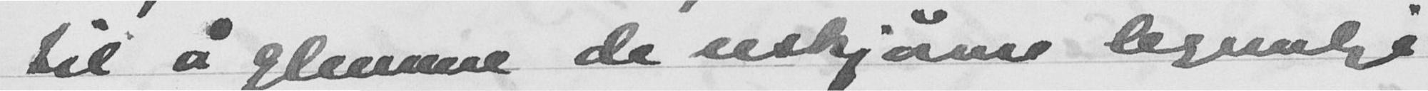til å glemme de uskjønne legemlige
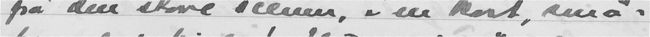på den store scenen, i en kort, små-
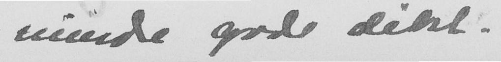mindre god dikt.
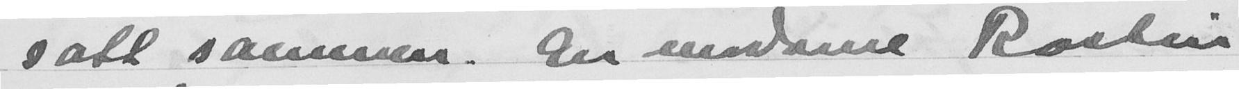satt sammen. En moderne Rastin
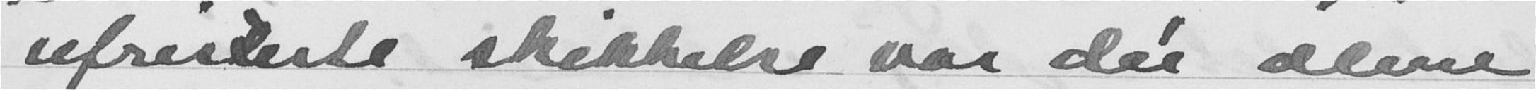ufriserte skikkelse var der alene
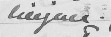linjene.
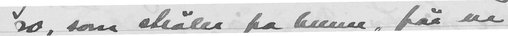ro, som stråler fra henne, får en
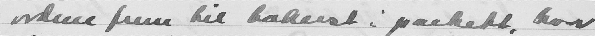ordene frem til bakerst i paskabt, hvor
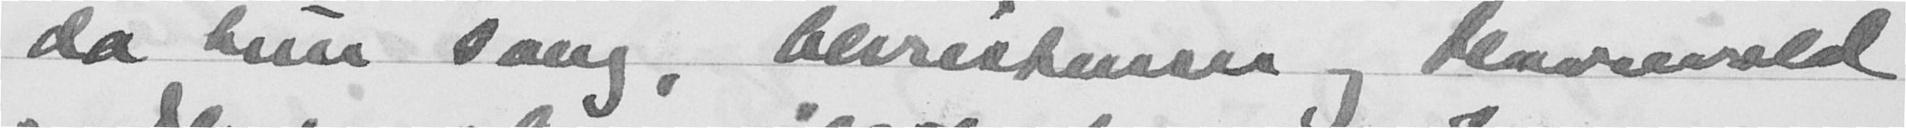da hun sang, berristenser og Havrevald
